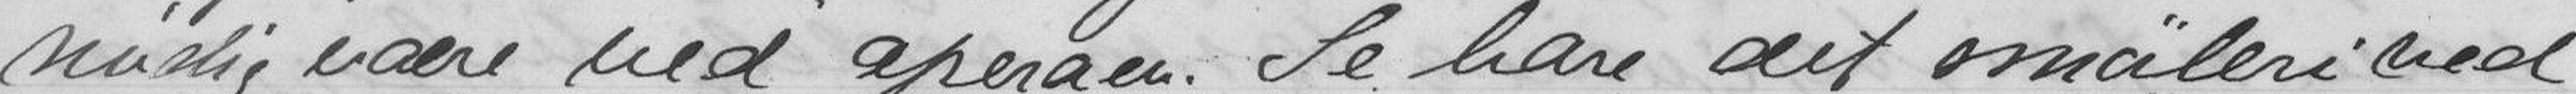nødig være ved operaen. Se bare han smäleri ved
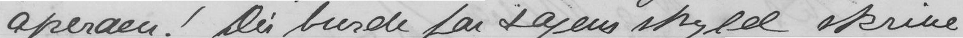operaen! Du burde for sagens skyld skrive
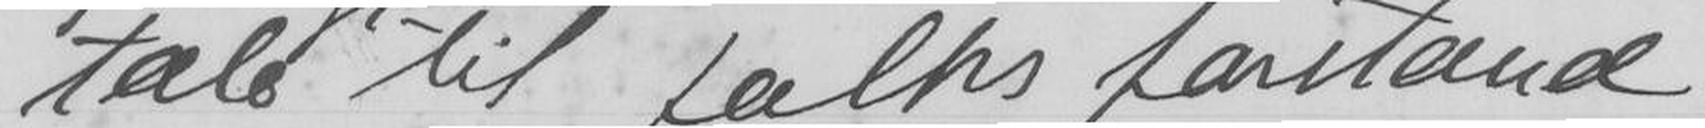tale til folks forstand.
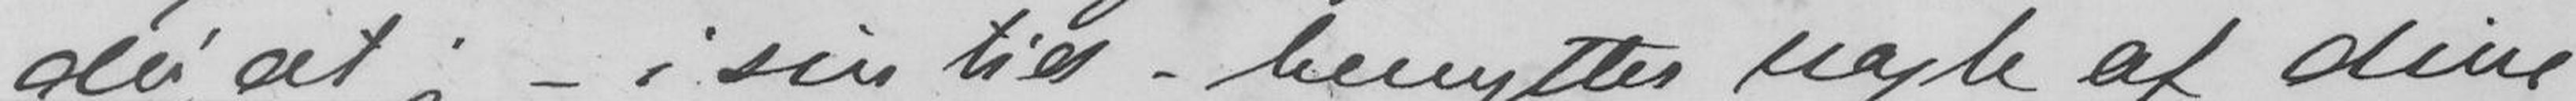du, at jeg - i sin tid - benyttes nogle af dine
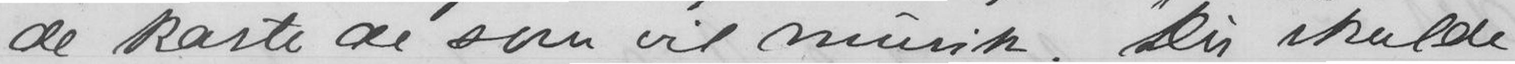de koste de som vil musik. Du skulde
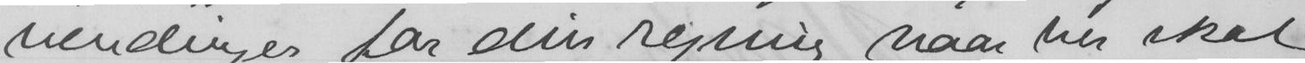vendinger for din regning, naar her skal
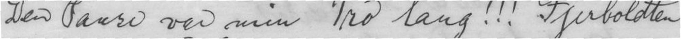Den Pause var min Tro lang!!! Fjerboldten
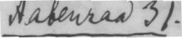Aabenraa 31.
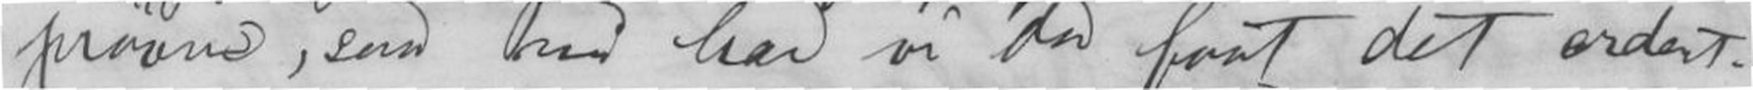prøven, saa nu har vi da faat det ordent-
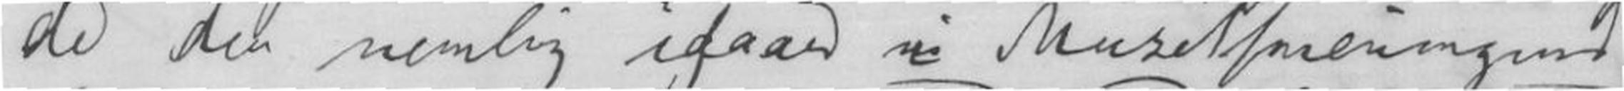de den nemlig igaar i Musikforeningen;
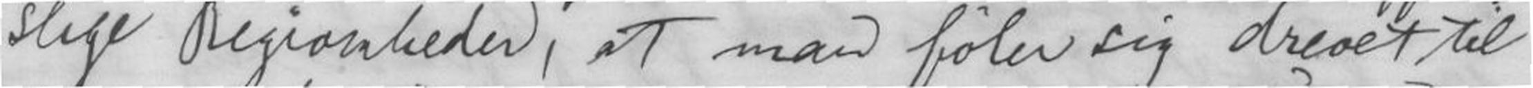slige Regionheder, at man føler sig drevet til
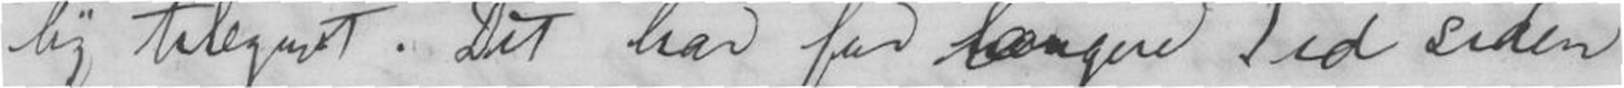lig tilegnet. Det har for længere Tid siden
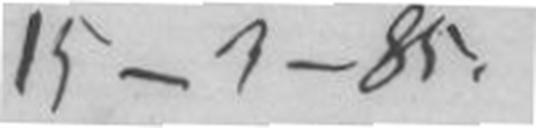15-1-85.
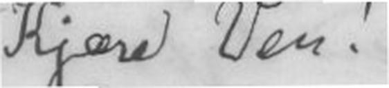Kjære Ven!
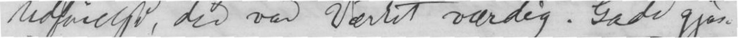Udførelse, der var Værket værdig. Gade gjor-
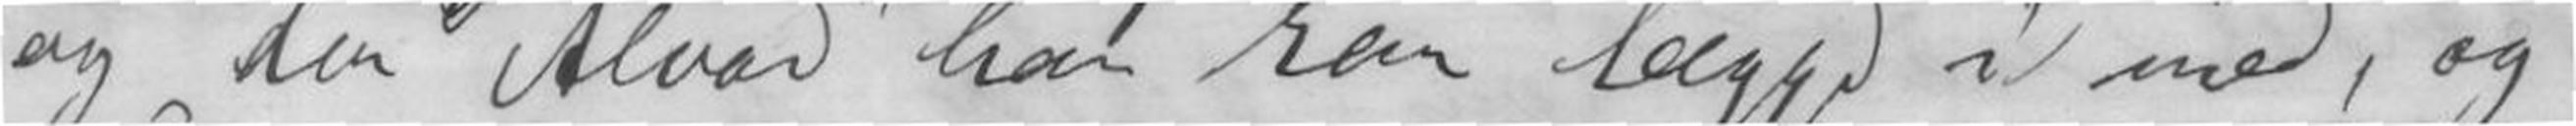og den alvor han kan lægge i med, og
